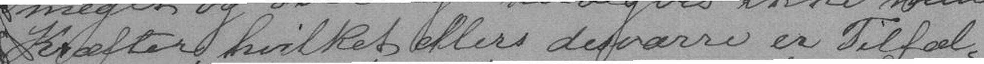Kræfter; hvilket ellers desværre er Tilfæl-
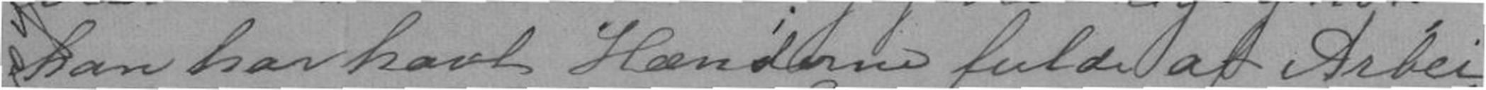han har havt Hænderne fulde af Arbei-
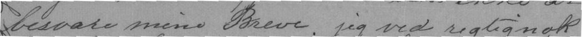besvare mine Breve; jeg ved rigtignok
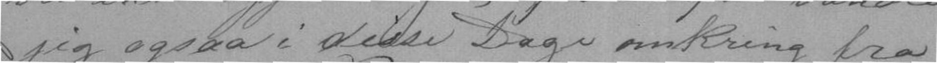jeg ogsaa i disse Dage omkring fra
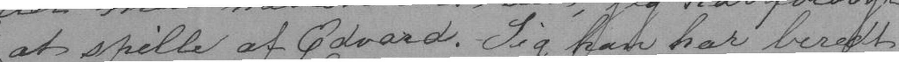at spille af Edvard. Sig han har beredt
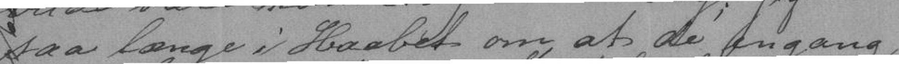saa længe i Haabet om at de engang
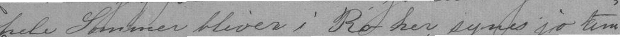hel Sommer bliver i Ro her synes jo tem-
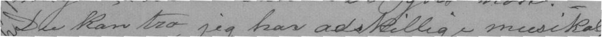Du kan tro jeg har adskillige musikal-
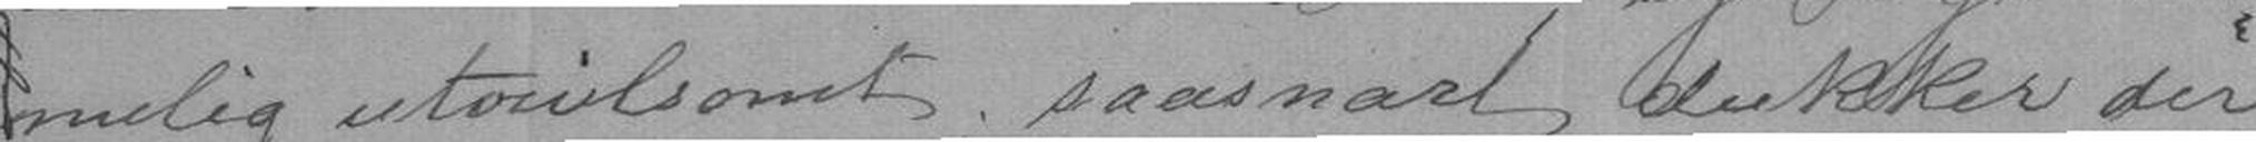melig utvivlsomt; saasnart dukker der
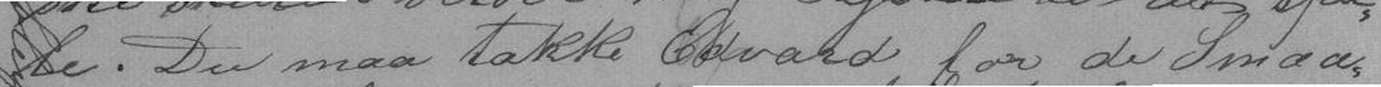le. Du maa takke Edvard for de Smaa-
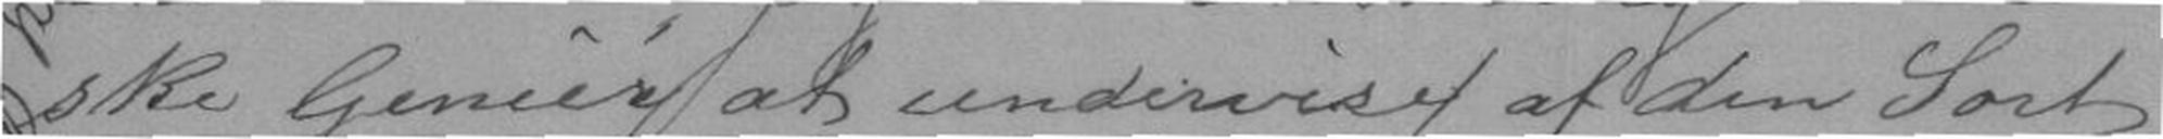ske Genier at undervise (af den Sort
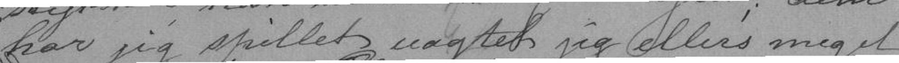har jeg spillet uagtet jeg ellers meget
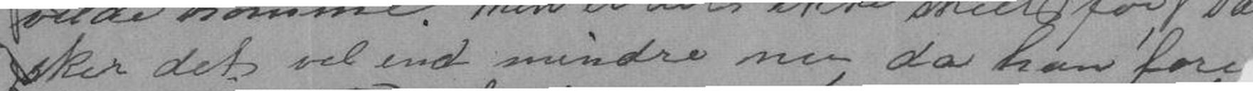sker det vel end mindre nu, da han fore
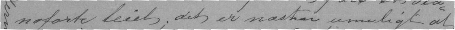noforte leiet; det er næsten umuligt at
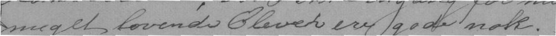meget lovende Elever ere gode nok. -
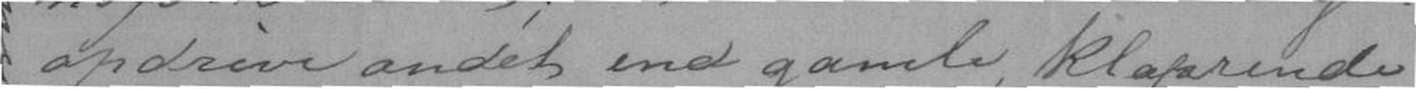opdrive andet end gamle, klaprende
